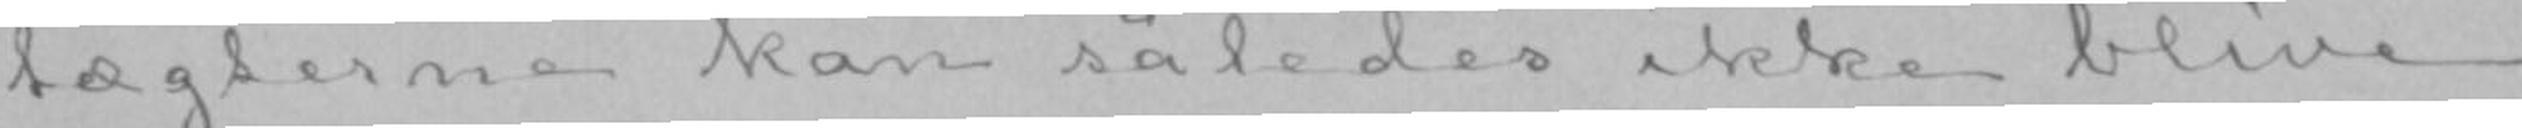tægterne kan således ikke blive
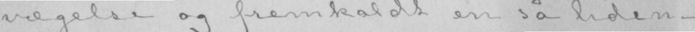vægelse og fremkaldt en så liden-
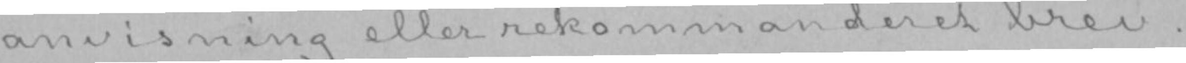anvisning eller rekommanderet brev.
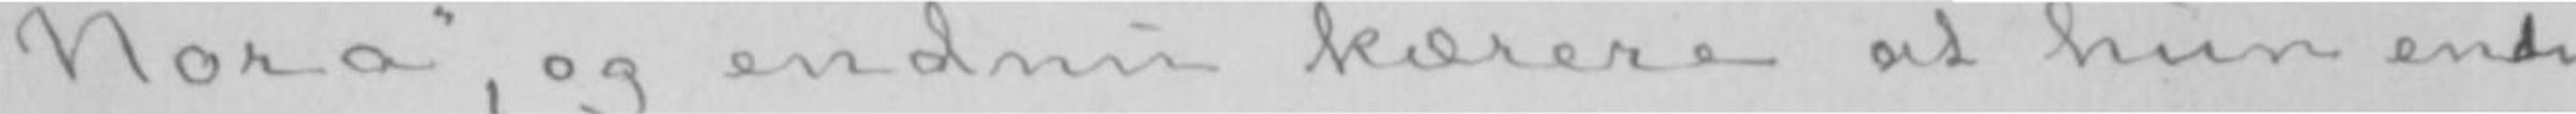«Nora», og endu kærere at hun ende-
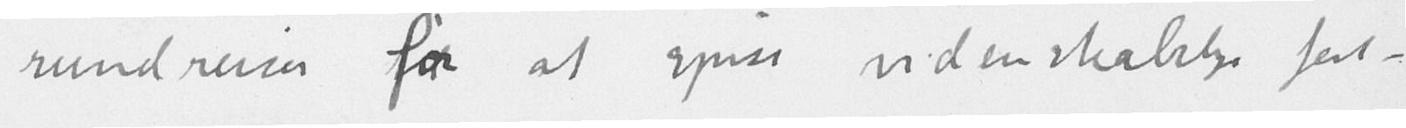rundreiser for at spise videnskabelige fest-
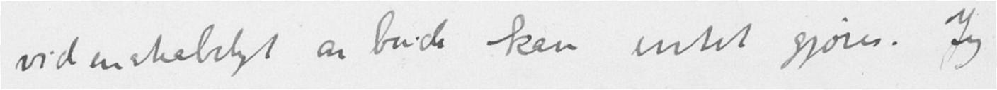videnskabeligt arbeide kan intet gjøres. Jeg
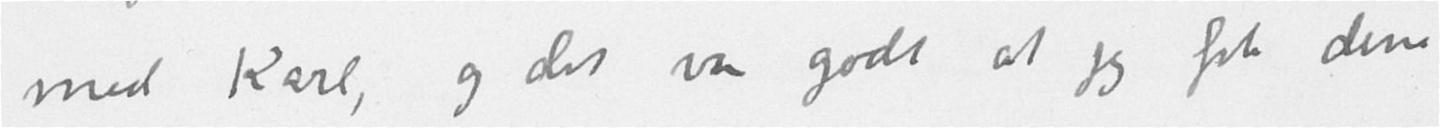med Karl, og det var godt at jeg fik dine
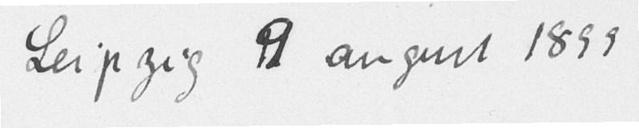Leipzig 9 august 1899
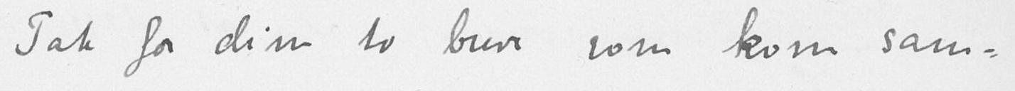Tak for dine to breve som kom sam-
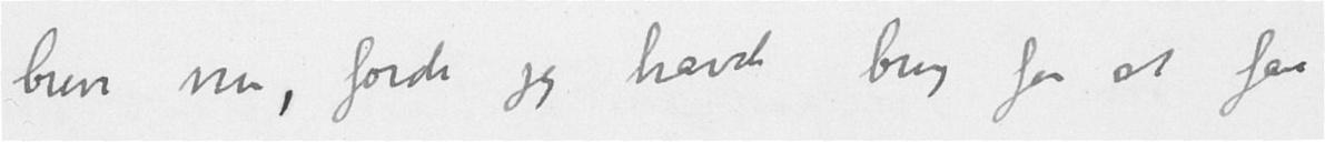breve nu, fordi jeg havde brug for at faa
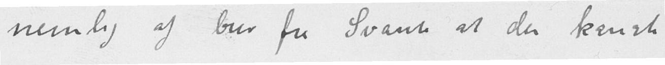nemlig af brev fra Svante at der kanske
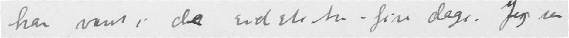har været i de sidste tre-fire dage. Jeg ser
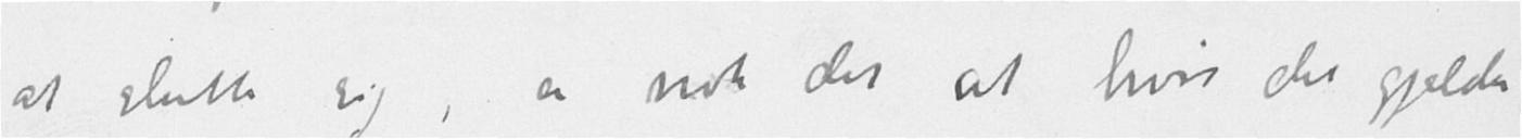at slutte sig, er nok det at hvis det gjelder
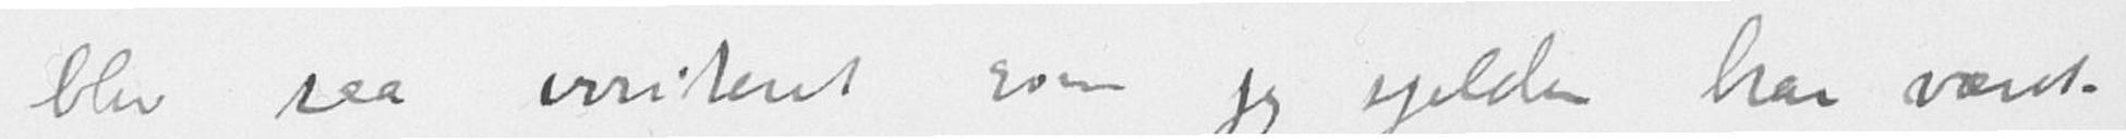blev saa irriteret som jeg sjelden har været.
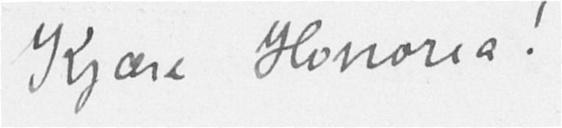Kjære Honoria!
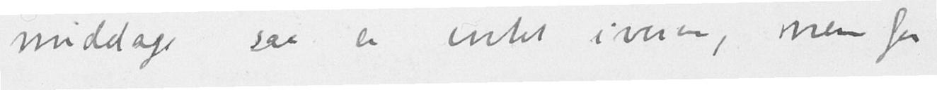middage saa er intet i veien, men for
